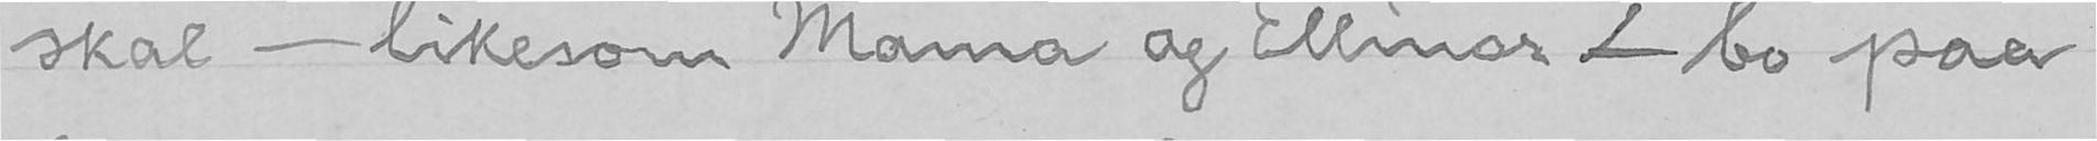skal - likesom Mama og Ellinor - bo paa
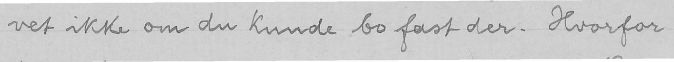vet ikke om du kunde bo fast der. Hvorfor
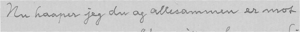Nu haaper jeg du og allesammen er mot
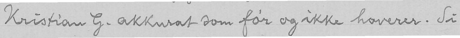Kristian G. akkurat som før og ikke hoverer. Si
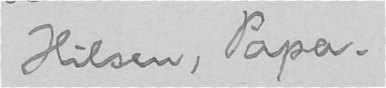Hilsen, Papa.
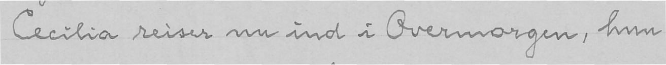Cecilia reiser nu ind i Overmorgen, hun
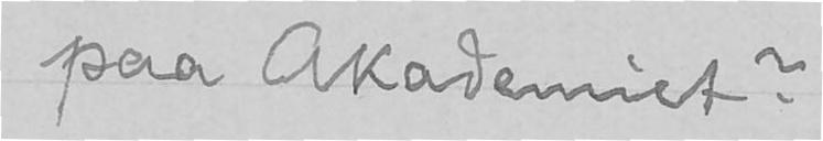paa Akademiet?
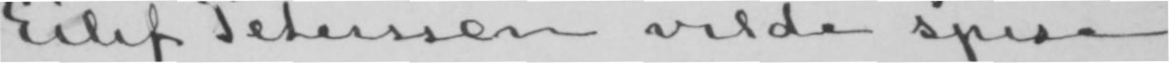Eilif Peterssen vilde spise
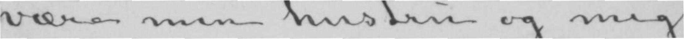være min hustru og mig
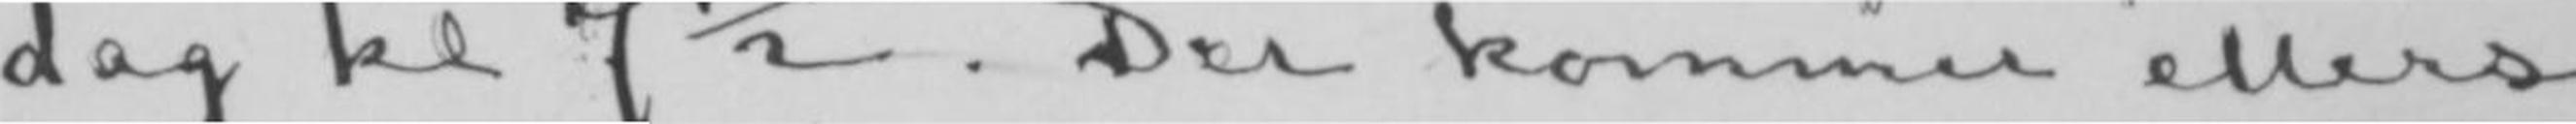dag kl 7½. Der kommer ellers
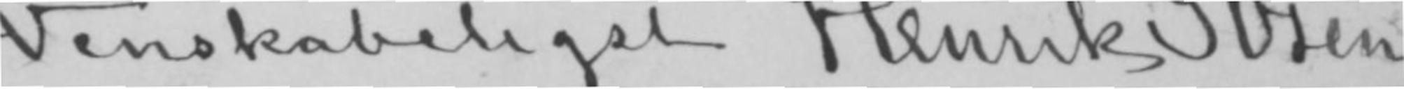Venskabeligst Henrik Ibsen
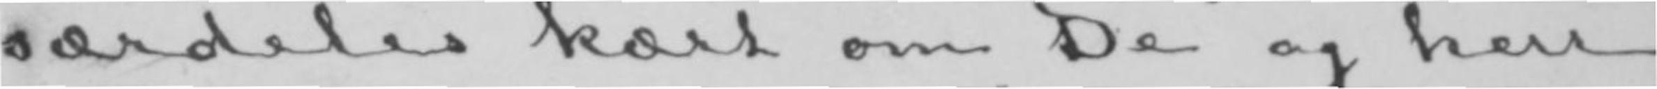særdeles kært om De og herr
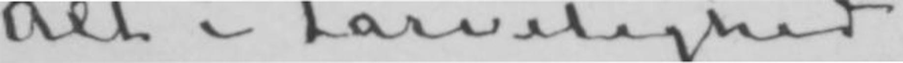Alt i tarvelighed.
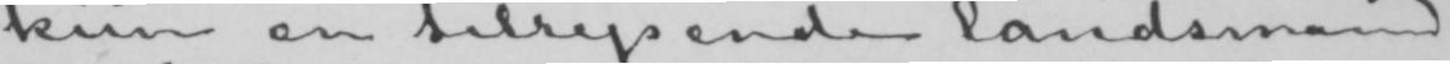kun en tilrejsende landsmand.
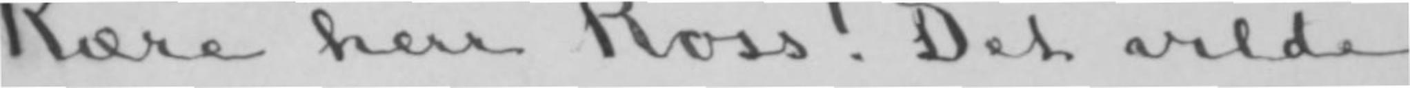Kære herr Ross! Det vilde
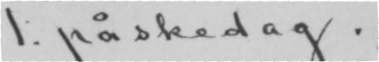1. påskedag.
